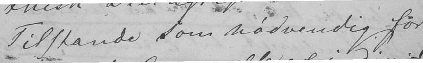Tilstande som nødvendig for
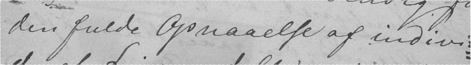den fulde Opnaaelse af individi-
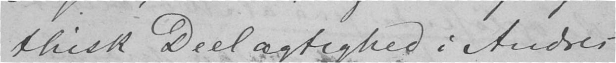thisk Deelagtighed i Andres
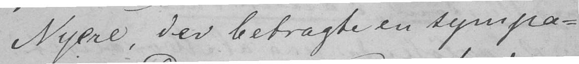Nyere, der betragte en sympa-
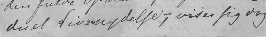duel Livsnydelse, viser sig dog
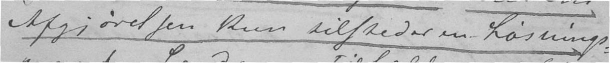Afgjørelsen kun tilsteder en Løsnings-
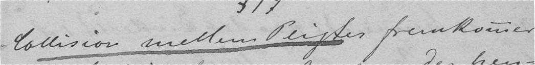Collision mellem Pligter forekommer,
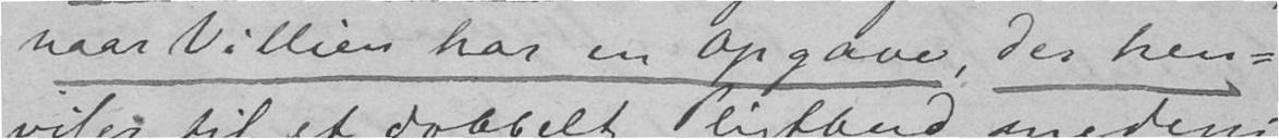naar Villien har en opgave, der hen-
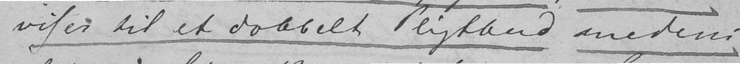viser til et dobbelt Pligtbud medens
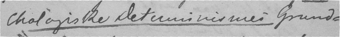chologiske Determinismes Grund-
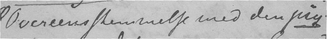Overeensstemmelse med den psy-
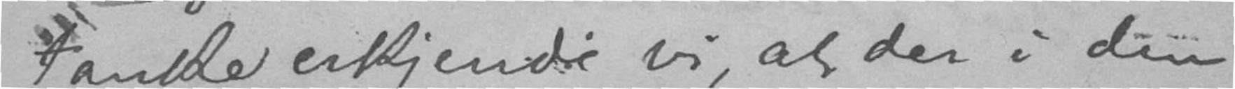tanke erkjende vi, at der i den
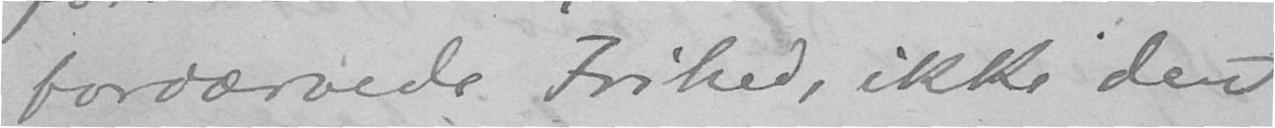fordærvede Frihed, ikke den
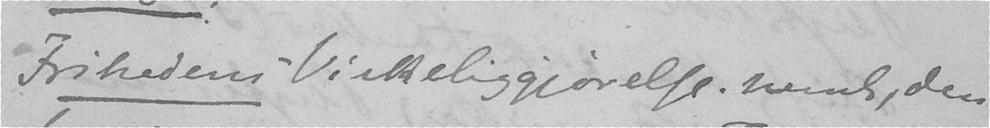Frihedens Virkeliggjørelse hen, den
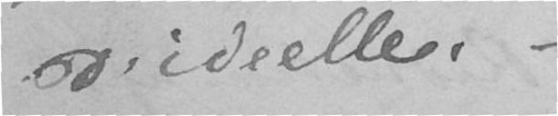ideelle. –
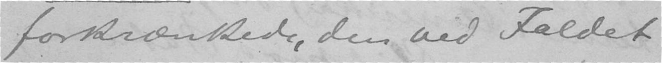forkrænkede, den ved Faldet
